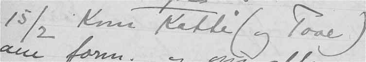15/2 Kom Katti (og Tove)
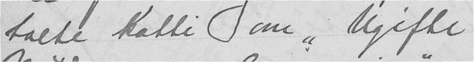talte Katti om "Ugifte
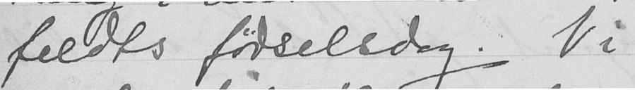feldts fødselsdag. Vi
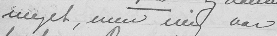meget, men mig var
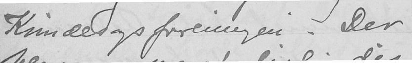Kvindesagsforeningen. Der
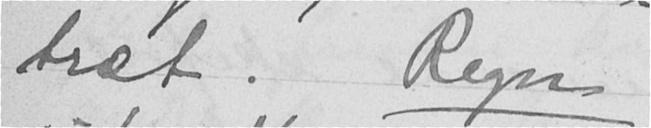træt. Regn
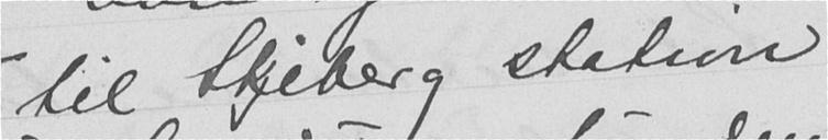til Skjeberg station
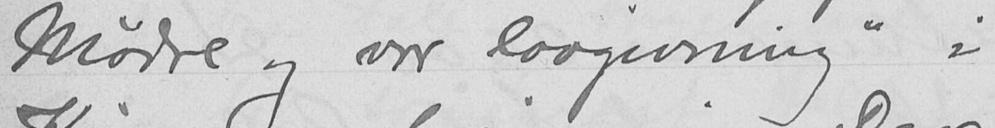Mødre og vor lovgivning" i
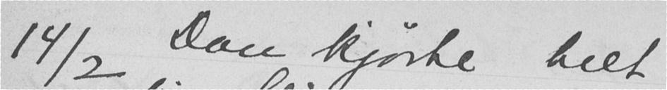14/2 Don kjørte helt
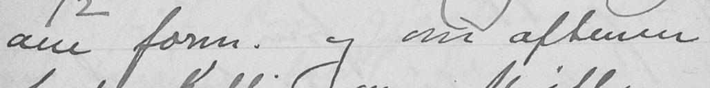om form. og om aftenen
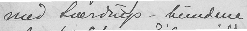med Sverdrups - bundene
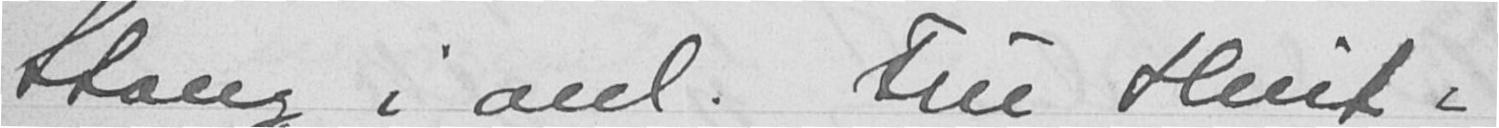Stang i anl. Fru Huit-
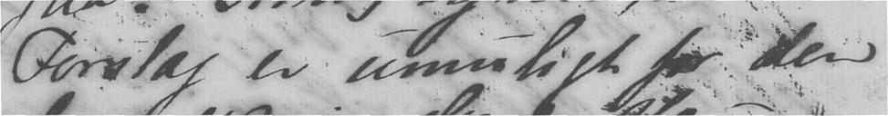Forslag er umuligt for den
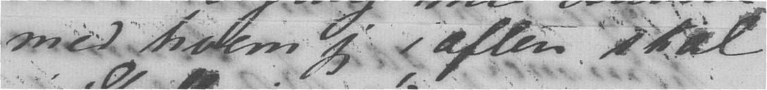med hvem jeg i aften skal
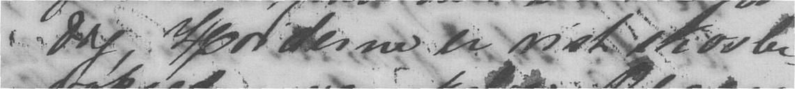dig, Høiderne er vist skovbe-
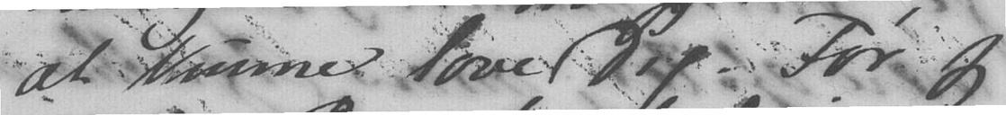at kunne love Dig. Før jeg
